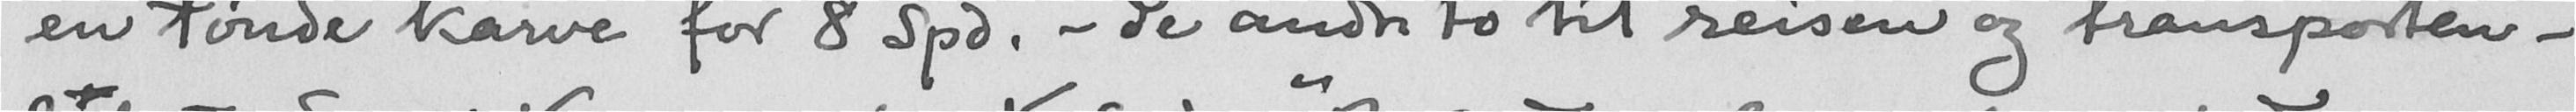en tønde karve for 8 spd. - de andre to til reisen og transporten-
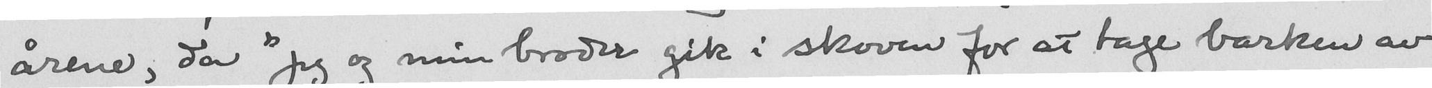årene, da "jeg og min broder gik i skoven for at tage barken av
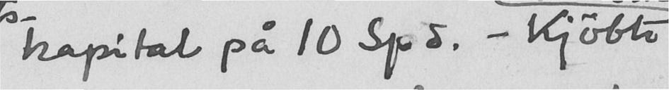kapital på 10 spd. - kjøbte
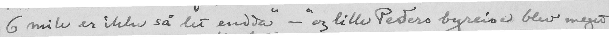6 mile er ikke så let endda" - "og lille Peders byreise blev meget
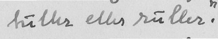tuller eller ruller."
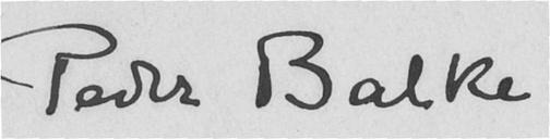Peder Balke
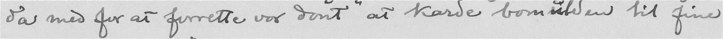da med for at forrette vor dont" at karde bomulden til fine
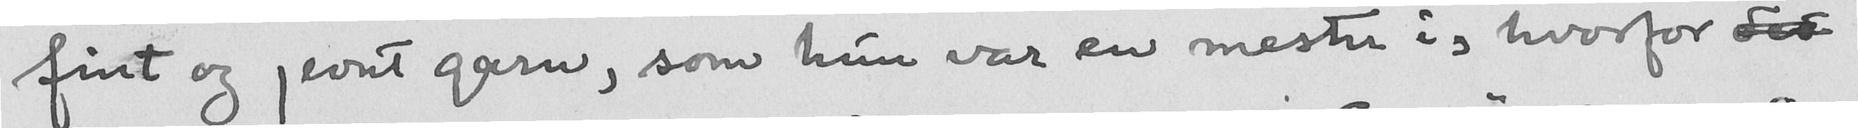fint og jevnt garn, som hun var en mester i, hvorfor det
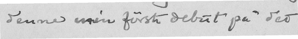denne min første debut på det
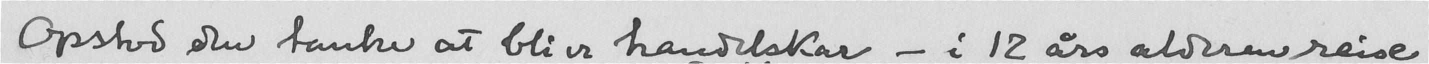Opstod den tanke at blive handelskar - i 12 års alderen reise
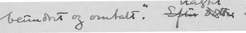beundret og omtalt." Efter dette
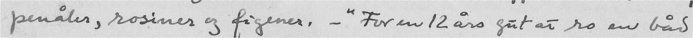penåler, rosiner og figener. -" For en 12 års gut at ro en båd
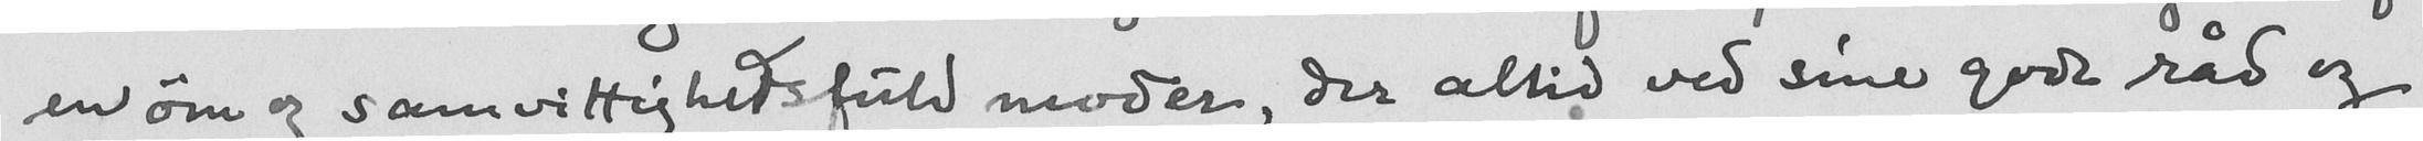en øm og samvittighetsfuld moder, der altid ved sine gode råd og
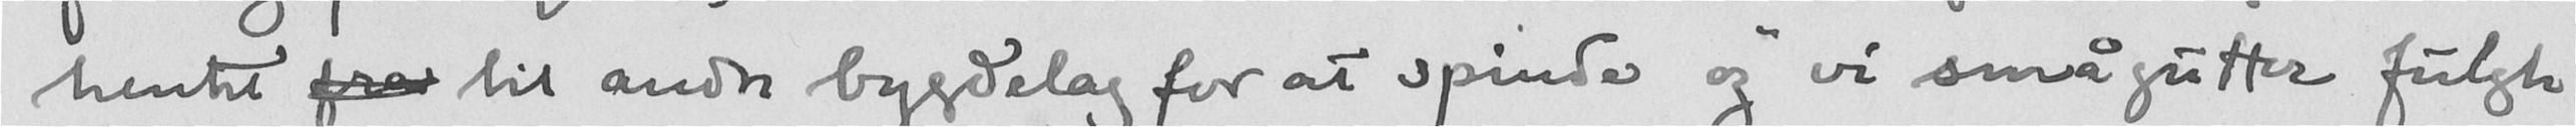hentet fra til andre bygdelag for at spinde og "vi smågutter fulgte
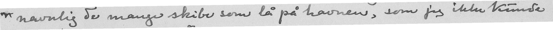nævnlig de mange skibe som lå på havnen, som jeg ikke kunde
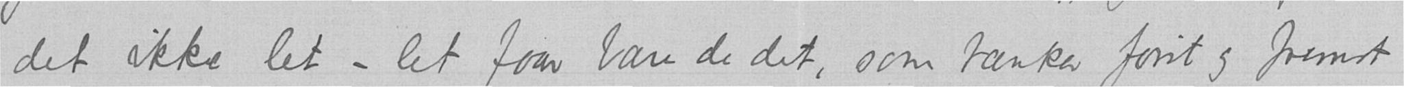det ikke let – let faar bare de det, som tænker først og fremst
